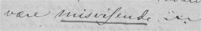være misvisende.
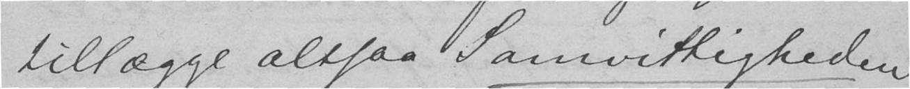tillægge altsaa Samvittigheden
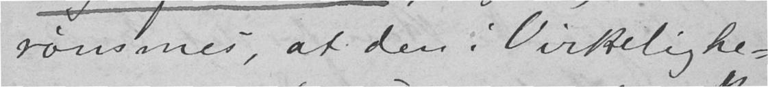rømmes, at den i Virkelighe-
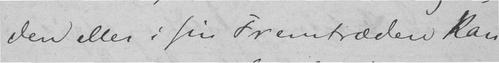den eller i sin Fremtræden kan
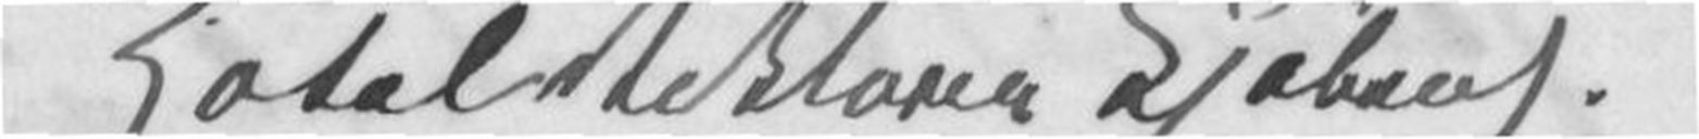Hotel Viktoria Kjøbenh.
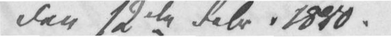Den 12te Febr. 1880.
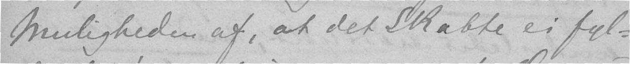Muligheden af, at det skabte ei fyl-
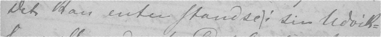Det kan enten stanse i sin Udvik-
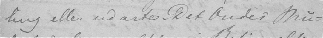ling eller udarte. Det Ondes Mu-
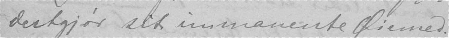destgjør sit immanente Øiemed.
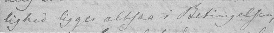lighed ligger altsaa i Betingelser,
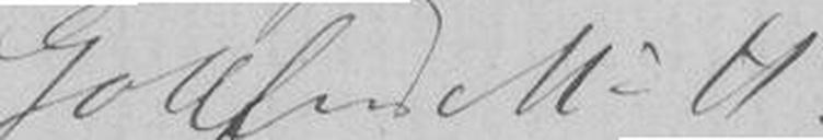Gottfred M=H.
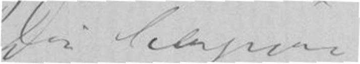Din hengivne
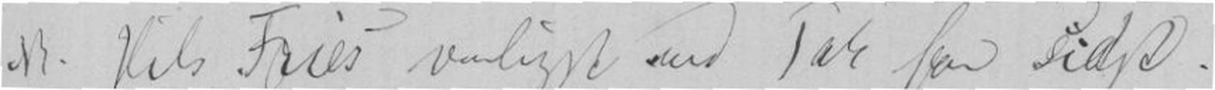NB. Hils Fries venligst med Tak for sidst.
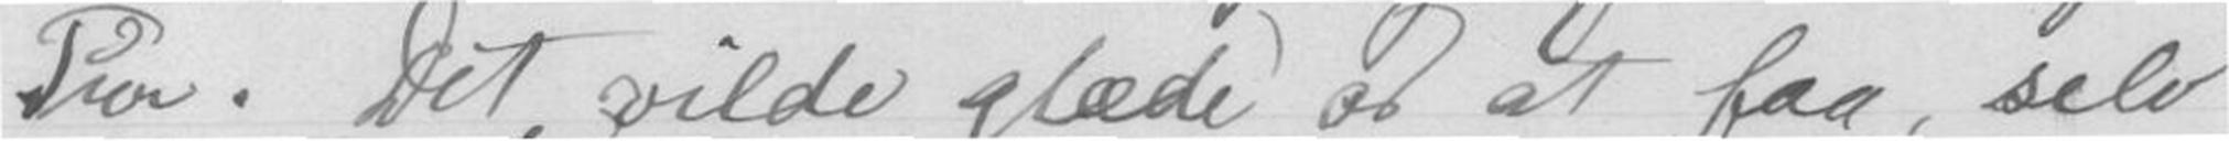Tur. Det vilde glæde os at faa, selv
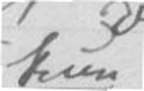kun
